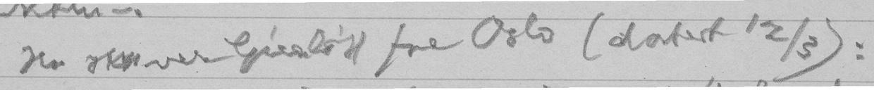Nu skriver Gierløff fra Oslo (datert 12/3):
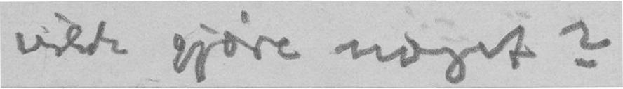vilde gjøre noget?
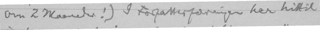om 2 Maaneder!) I Forfatterforeningen har hittil
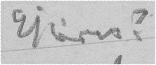gjøres!
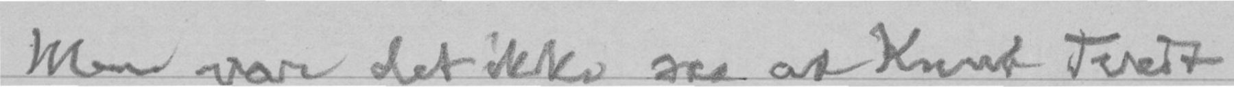Men var det ikke saa at Knut Tvedt
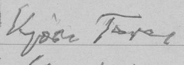Kjære Tore,
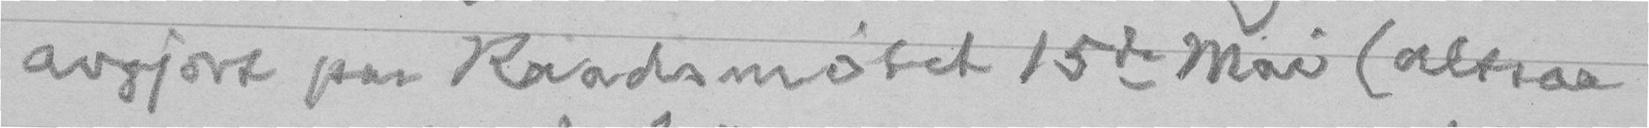avgjort paa Raadsmøtet 15de Mai (altsaa
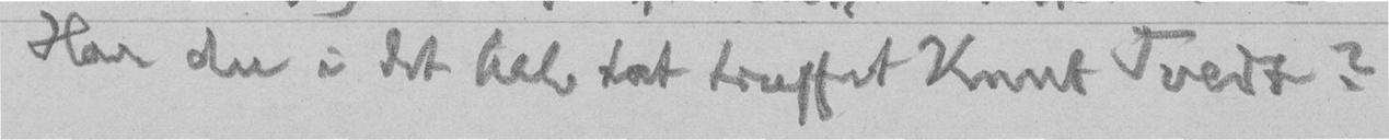Har du i det hele tat truffet Knut Tvedt?
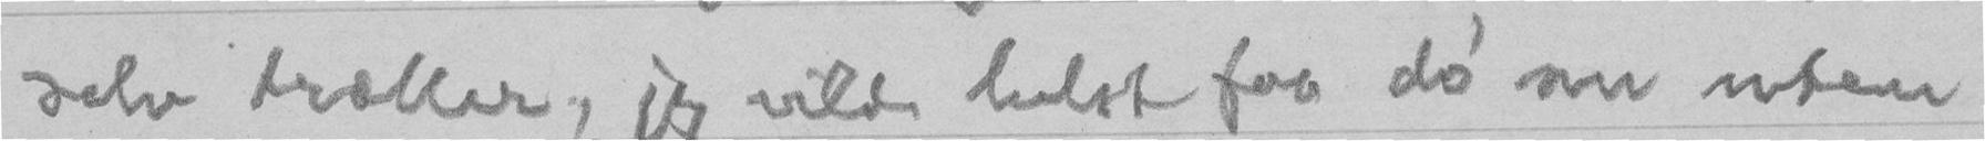selv træller, jeg vilde helst faa dø nu uten
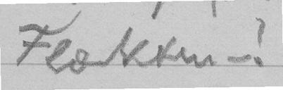Flækken.!
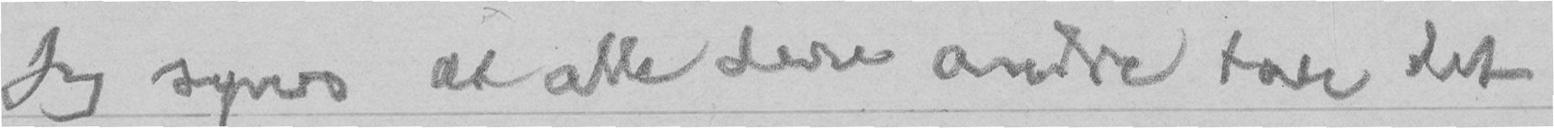Jeg synes at alle dere andre tar det
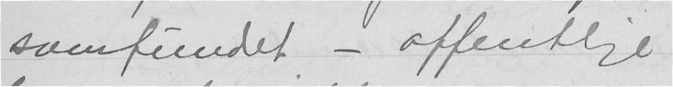samfundet - offentlige
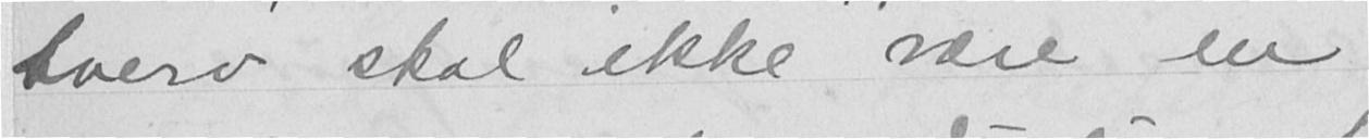hverv skal ikke være en
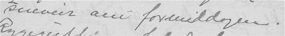sneveir om formiddagen.
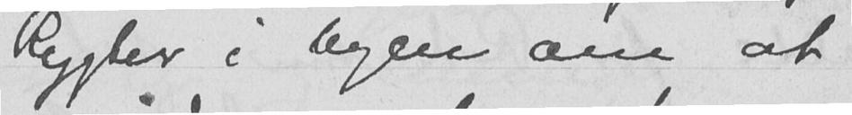Rygter i byen om at
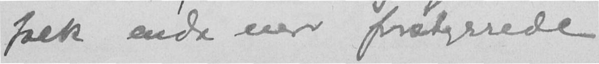folk enda mer forstyrrede
